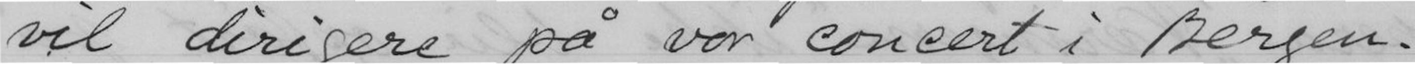vil dirigere på vor concert i Bergen.
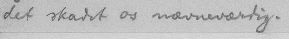det skadet os nævneværdig.
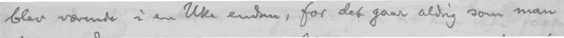blev værende i en Uke endnu, for det gaar aldrig som man
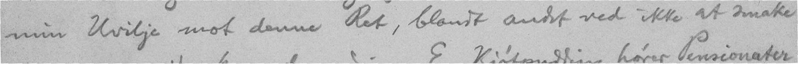min Uvilje mot denne Ret, blandt andet ved ikke at smake
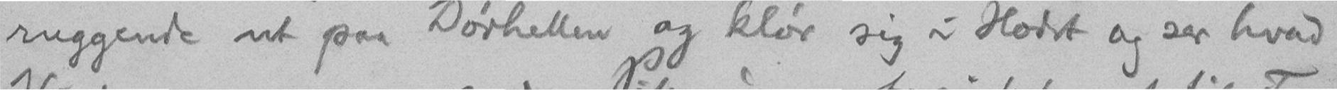ruggende ut paa Dørhellen og klør sig i Hodet og ser hvad
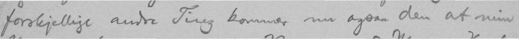forskjellige andre Ting kommer nu ogsaa den at min
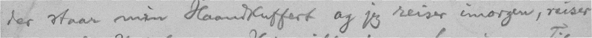der staar min Haandkuffert og jeg reiser imorgen, reiser
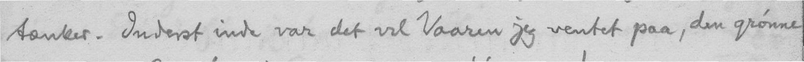tænker. Inderst inde var det vel Vaaren jeg ventet paa, den grønne
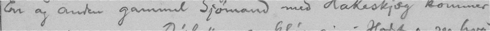En og anden gammel Sjømand med Hakeskjæg kommer
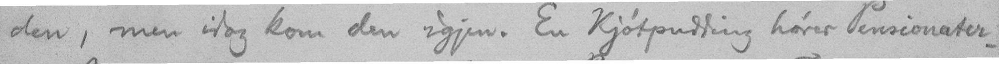den, men idag kom den igjen. En Kjøtpudding hører Pensionater-
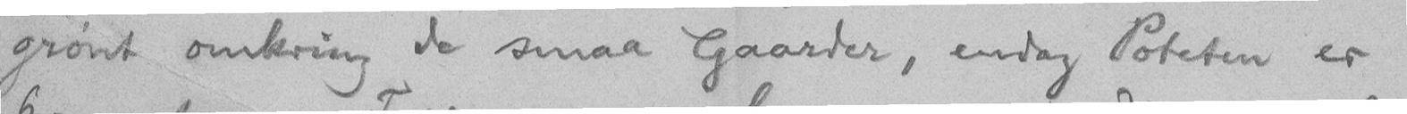grønt omkring de smaa Gaarder, endog Poteten er
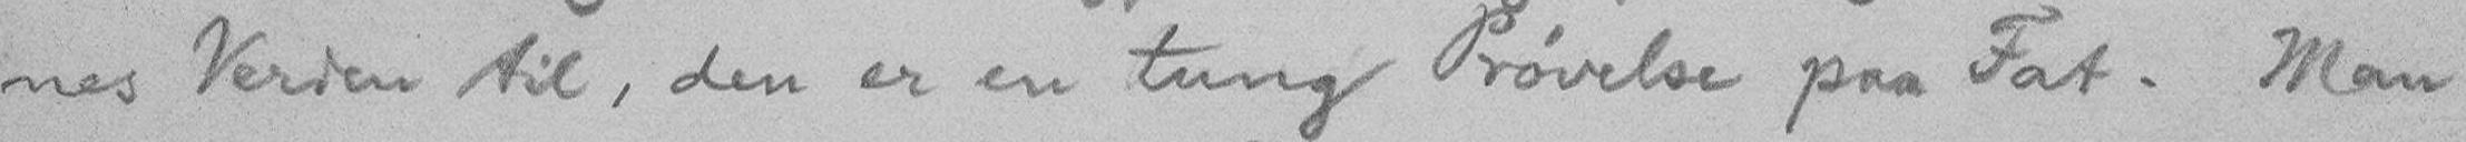nes Verden til, den er en tung Prøvelse paa Fat. Man
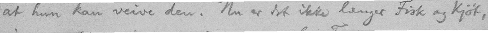at hun kan veive den. Nu er det ikke længer Fisk og Kjøt,
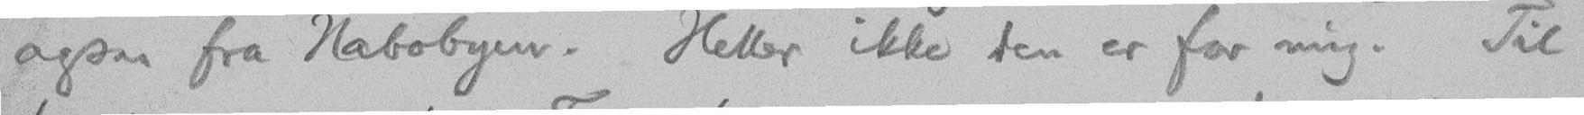ogsaa fra Nabobyen. Heller ikke den er for mig. Til
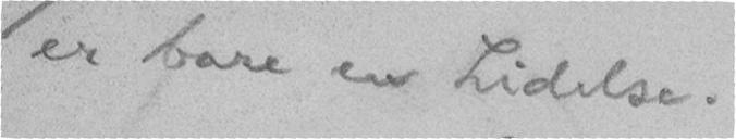er bare en Lidelse.
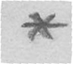*
